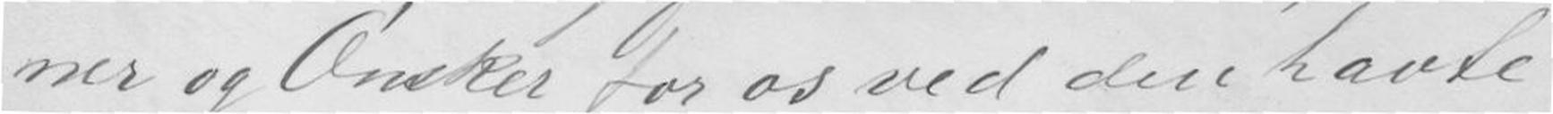ner og Ønsker for os ved dere havte
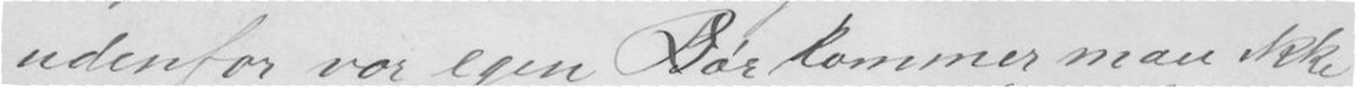udenfor vor egen Dør kommer man ikke
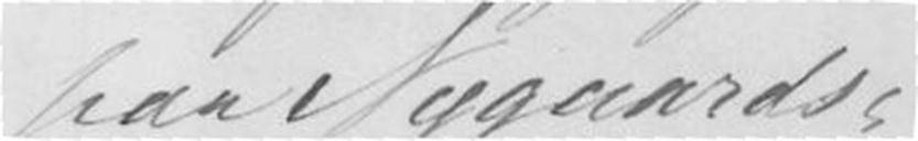paa Nygaards-
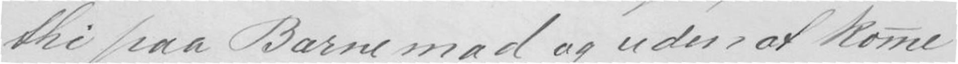thi paa Barne mad og uden at kome
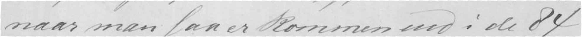naar man faaer kommen med i de 84
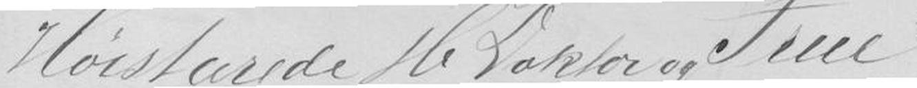Høystærede Hr. Doktor og Frue
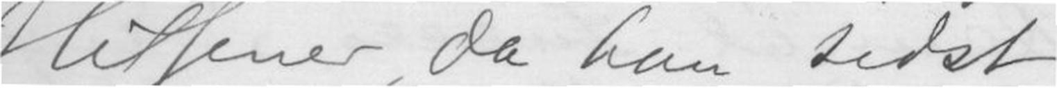Hisener, da han sidst
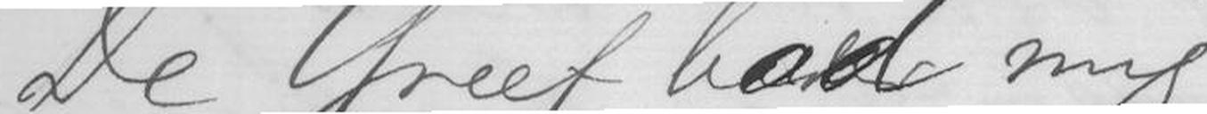De Greef bad mig
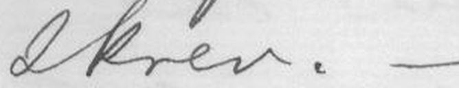skrev. -
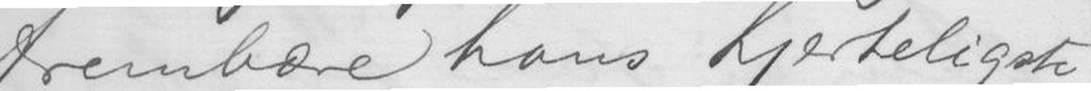frembære hans hjerteligste
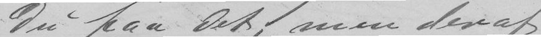Du faa det; men deraf
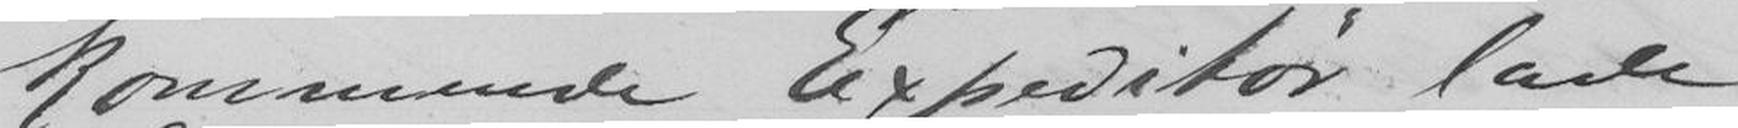kommende Expeditør lade
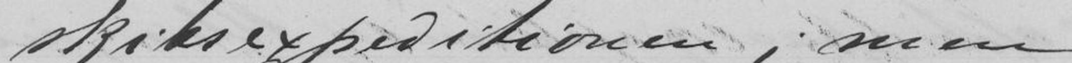skibsexpeditionen; men
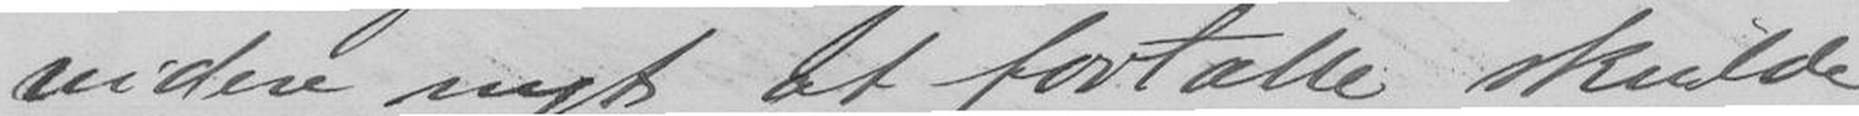videre nyt at fortælle skulde
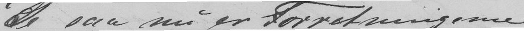Se saa nu er Forretningerne
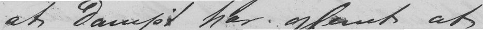at Damp: har glemt at
